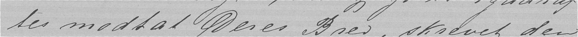tes modtat Deres Brev, skrevet den
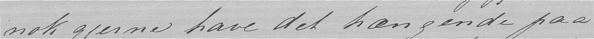nok gjerne have det hængende paa
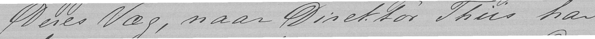Deres Væg, naar Direktør Thiis har
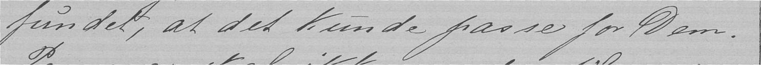fundet, at det kunde passe for Dem.
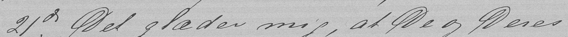21de. Det glæder mig at De og Deres
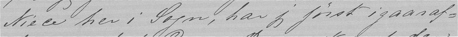Niece her i Sogn, har jeg først igaaraf-
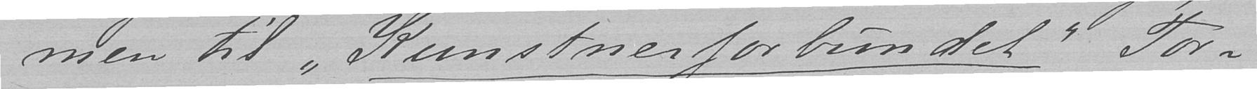men til "Kunstnerforbundet" Tor-
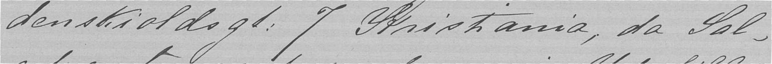denskioldsgt. 7 Kristiania, da Sal-
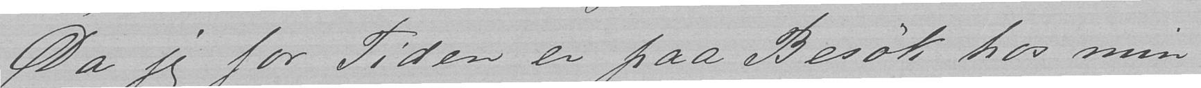Da jeg for Tiden er paa Besøk hos min
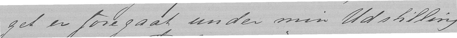get er foregaat under min Udstilling
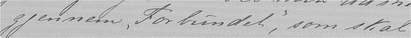gjennem Forbundet, som skal
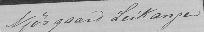Njøsgaard Leikanger
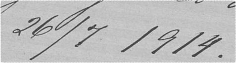26/7 1914.
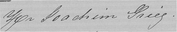Hr Joachim Grieg.
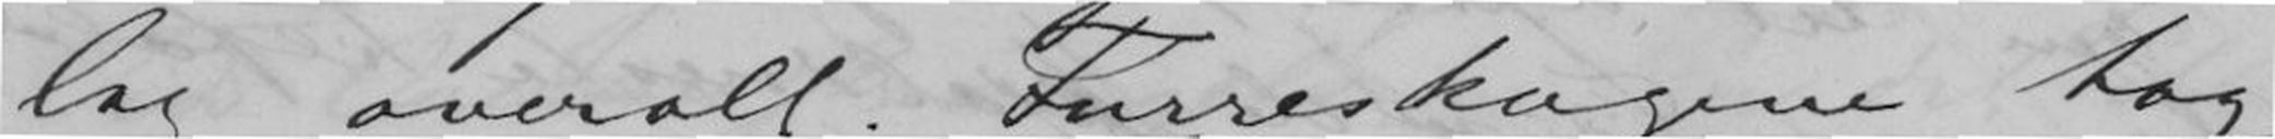lag overslt. Furreskogene tog
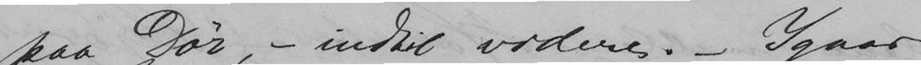paa Dør, - indtil videre. - Igaar
